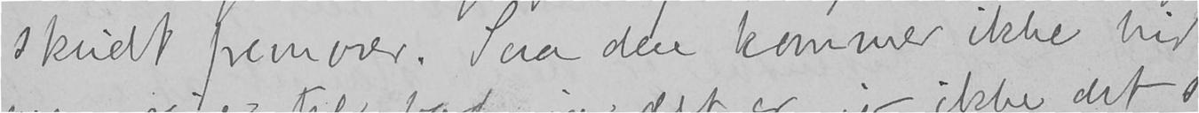skridt fremover. Saa dere kommer ikke hid
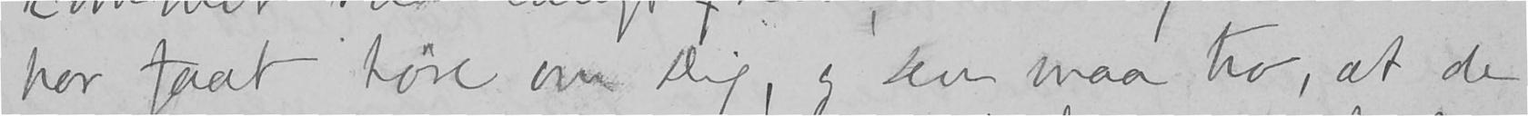har faat høre om Dig, og Du maa tro, at de
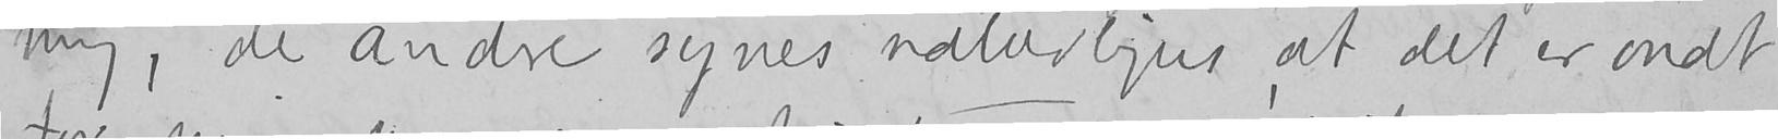mig, de andre synes naturligvis, at det er ondt
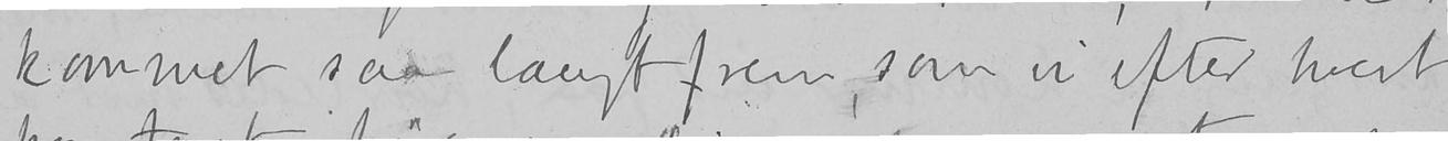kommet saa langt frem, som vi efter hvert
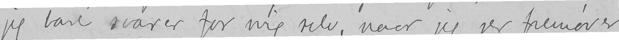jeg bare svarer for mig selv, naar jeg ser fremover
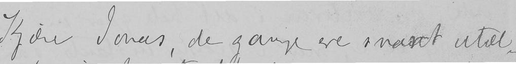Kjære Jonas, de gange ere snart utæl-
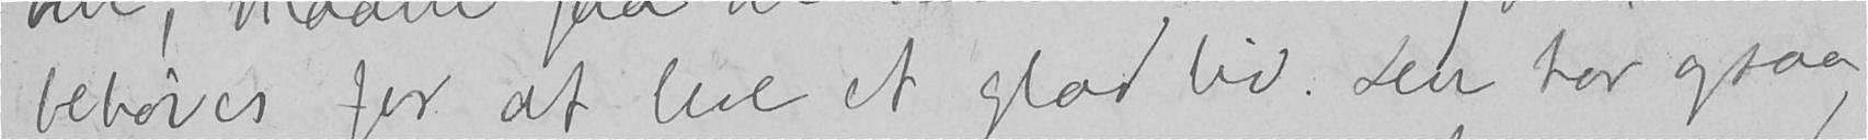behøves for at leve et glad liv. Du har ogsaa
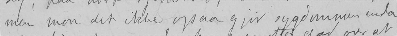men mon det ikke ogsaa gjør sygdommen enda
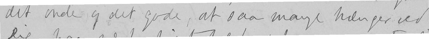det onde og det gode, at saa mange hænger ved
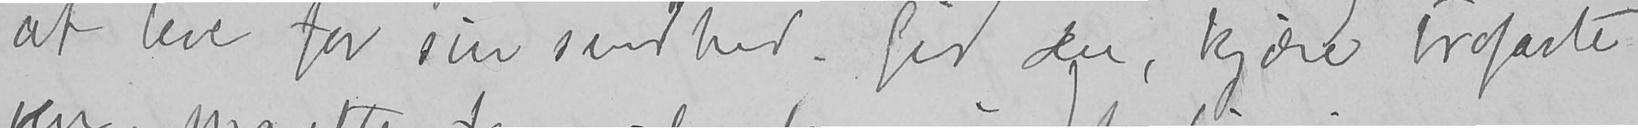at leve for sin sundhed. Gid Du, kjære trofaste
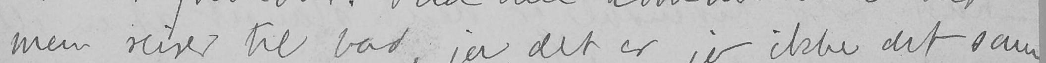men reiser til bad, ja det er jo ikke det sam-
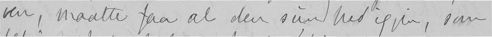ven, maatte faa al den sundhed igjen, som
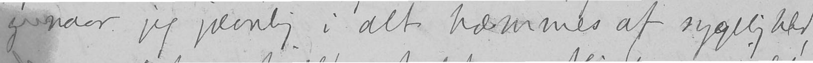og naar jeg jævnlig i alt hæmmes af sygelighed,
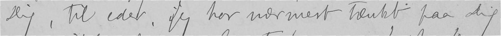Dig, til eder, jeg har nærmest tænkt paa Dig
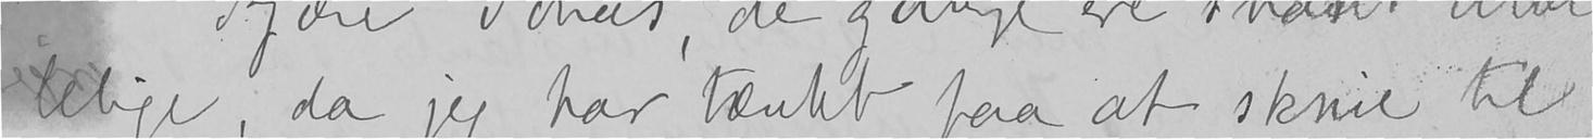lelige, da jeg har tænkt paa at skrive til
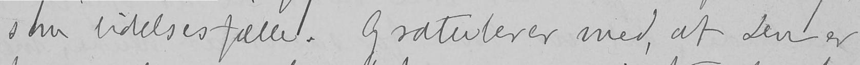som lidelsesfælle. Gratulerer med, at Du er
